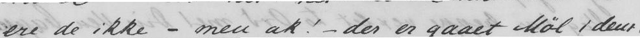ere de ikke - men ak! - der er gaaet Møl i dem.
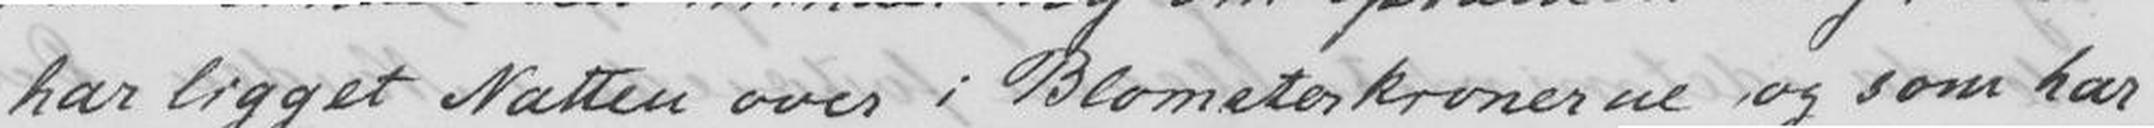har ligget Natten over i Blomsterkronerne og som har
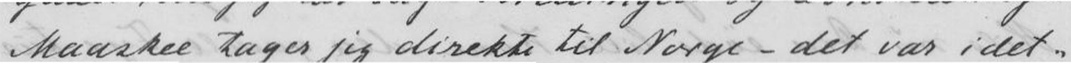Maaskee tager jeg direkte til Norge - det var idet-
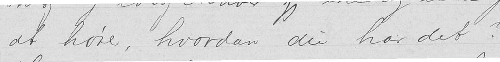at høre, hvordan du har det?
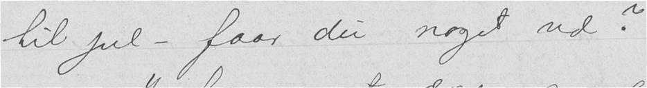til jul - faar du noget ud?
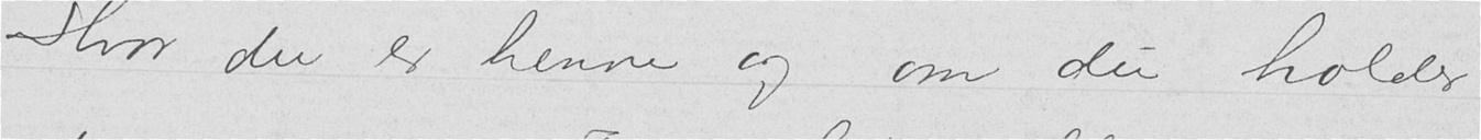Hvor der er henne og om du holder
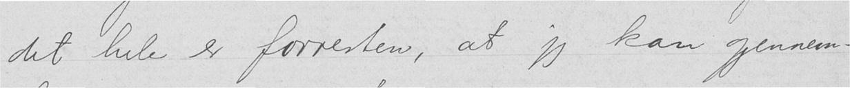det hele er forresten, at jeg kan gjennem-
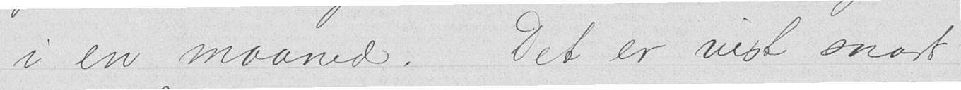i en maaned. Det er vist snart
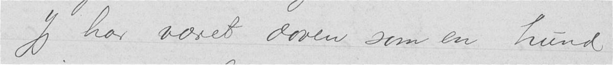Jeg har været doven som en hund
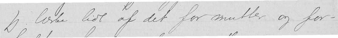Jeg leste lidt af det for mutter og for-
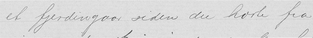et fjerdingaar siden du hørte fra
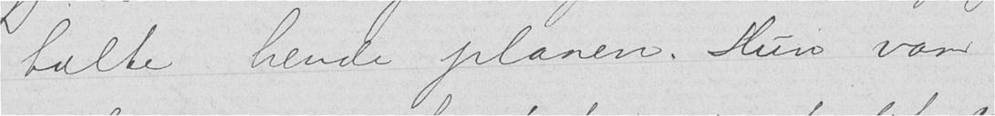talte hende planen. Hun var
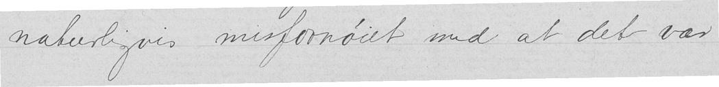naturligvis misfornøiet med at det var
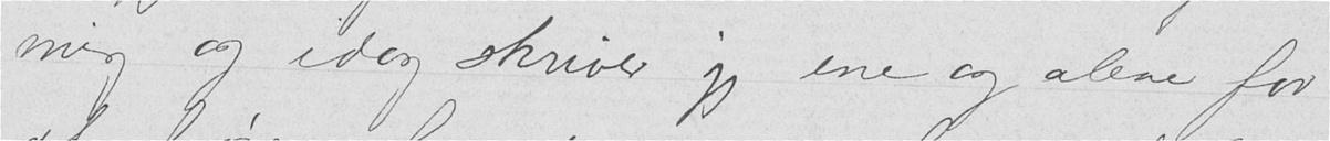mig og idag skriver og ene og alene for
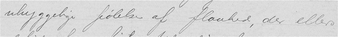uhyggelige følelse af flauhed, der ellers
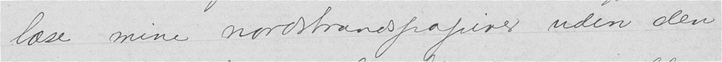læse mine nordstrandspapirer uden den
